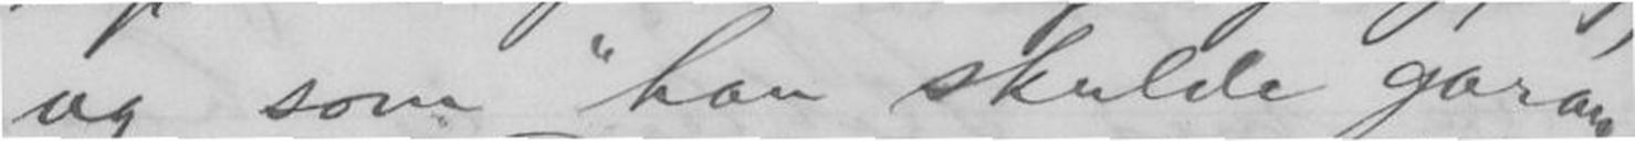og som "han skulde garan-
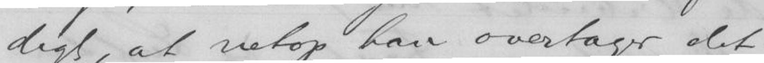digt, at netop han overtager det
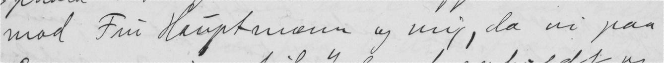mod Fru Hauptmann og mig, da vi paa
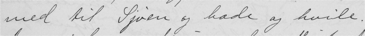med til Sjøen og bade og hvile.
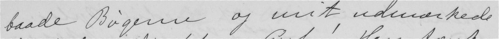baade Bøgerne og mit udmærkede
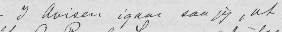I avisen igaar saa jeg, at
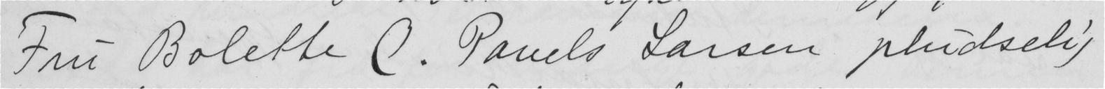Fru Bolette C. Pavels Larsen pludselig
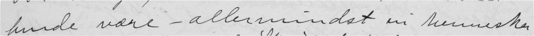kunde være - allermindst vi Mennesker
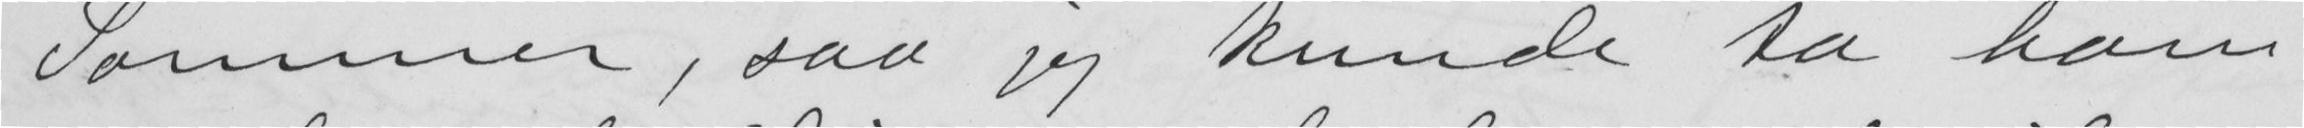Sommer, saa jeg kunde ta ham
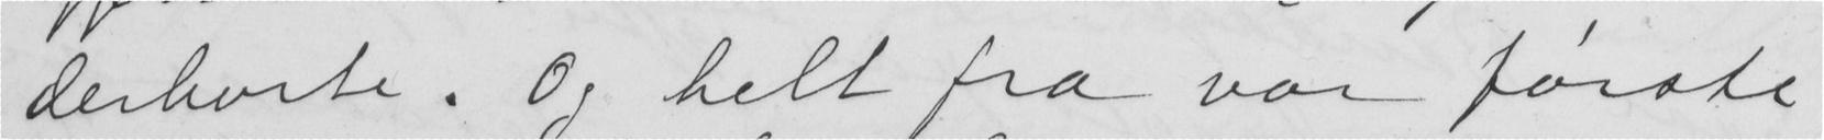derborte. Og helt fra vor første
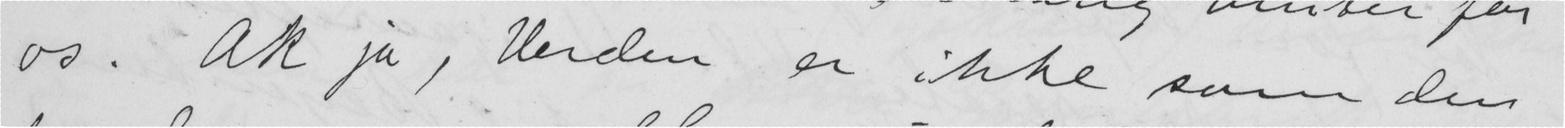os. Ak ja, Verden er ikke som den
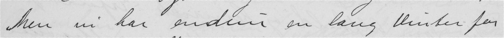Men vi har endnu en lang Vinter for
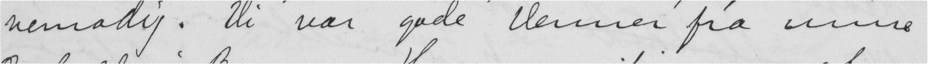vemodig. Vi var gode Venner fra mine
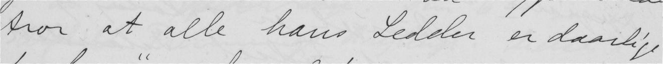tror at alle hans Ledder er daarlige
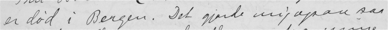er død i Bergen. Det gjorde mig ogsaa saa
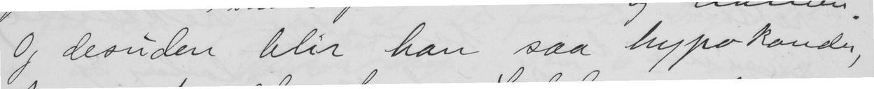Og desuden blir han saa hypokander,
